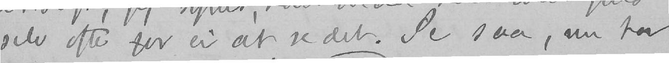selv ofte for ei at se det. Se saa, nu har
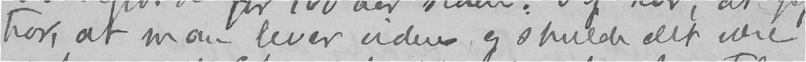tror, at man lever videre og skulde det være
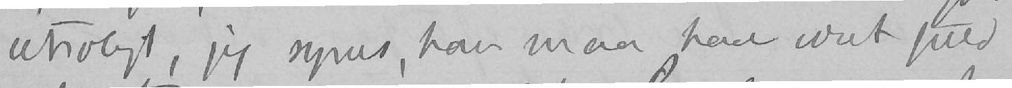utroligt, jeg synes, han maa have været fuld
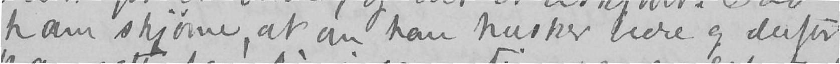ham skjønne, at om han husker bedre og derfor
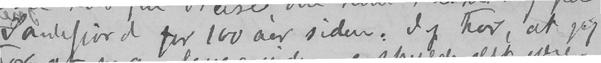Sandefjord for 100 aar siden. Jeg tror, at jeg
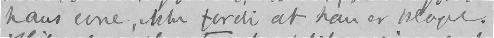hans evne, ikke fordi at han er klogere.
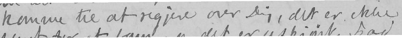komme til at regjere over Dig, det er ikke
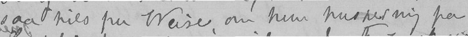saa hils fru Weise, om hun husker mig fra
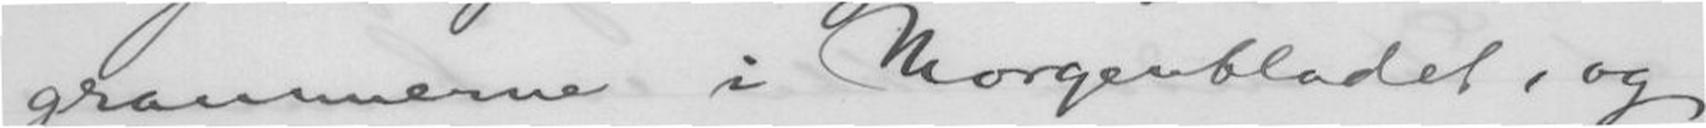grammerne i Morgenbladet, og
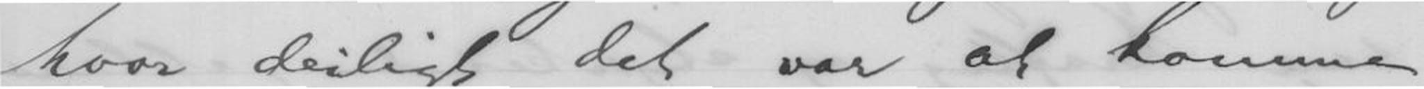hvor deiligt det var at komme
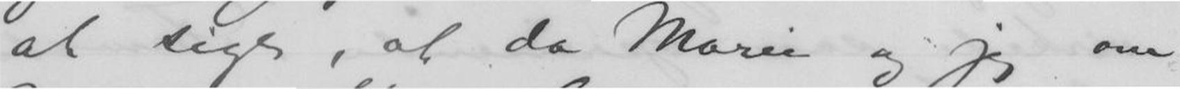at sige, at da Marie og jeg om
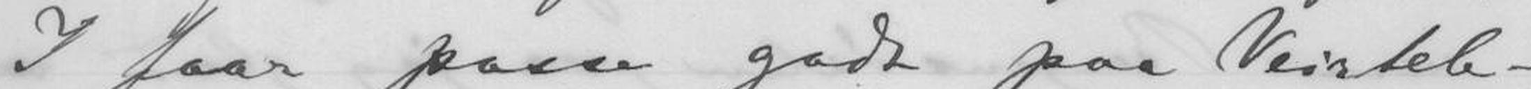I faar passe godt paa Veirtele-
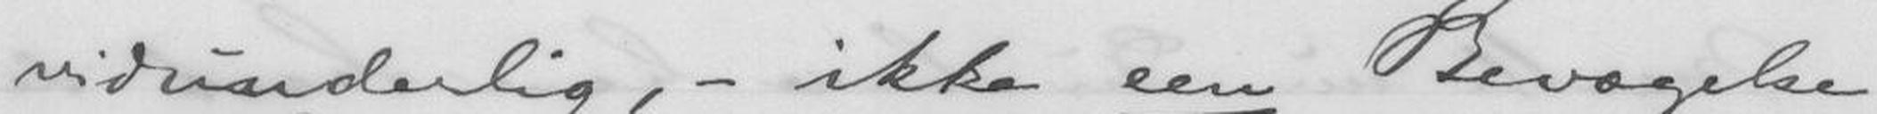vidunderlig, - ikke een Bævegelse
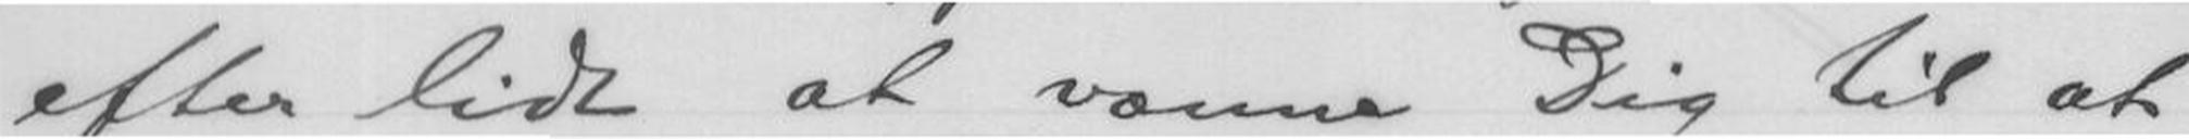efter lidt at vænne Dig til at
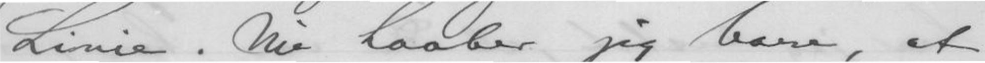Linie. Nu haaber jeg bare, at
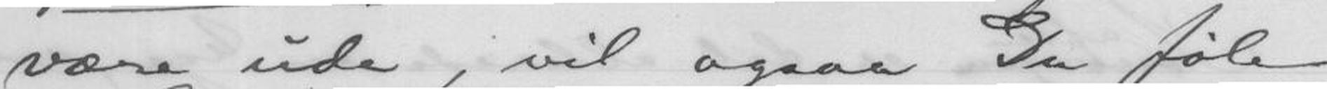være ude, vil ogsaa Du føle
